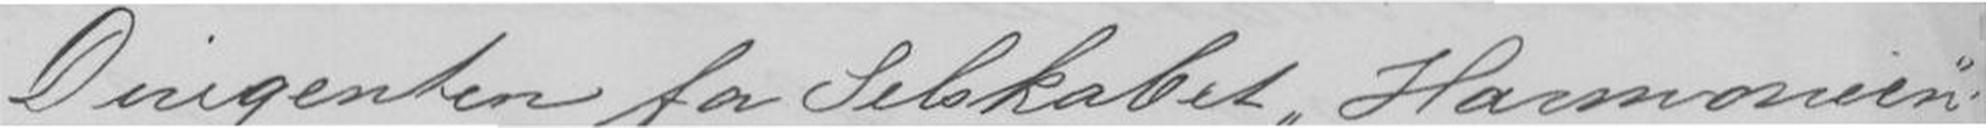Dirigenten for Selskabet "Harmonien"
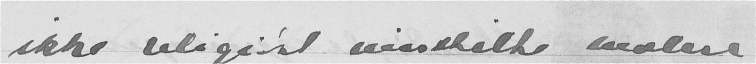ikke religiøst innstilte malere
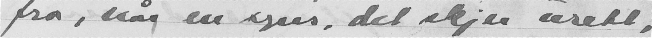fra, når en syns, det skjer urett,
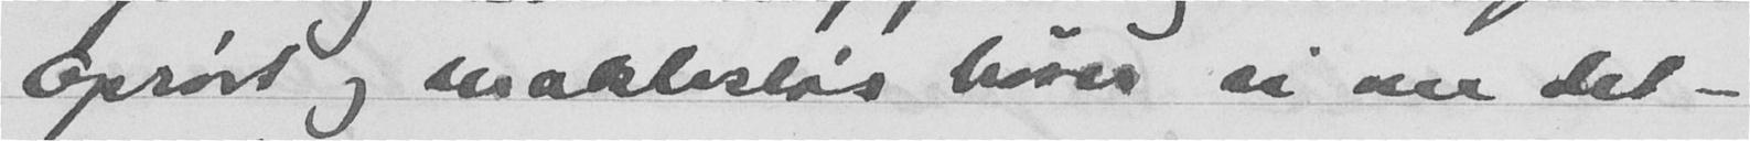Oprørt og maktesløs hører vi om det -
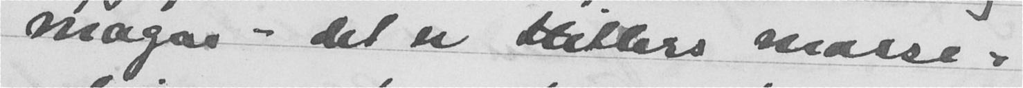magen - det er Hitlers masse-
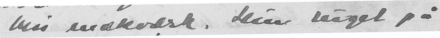heri enkværk. Hun ruget på
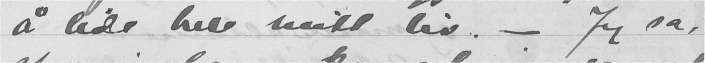å lide hele mitt liv. - Jeg sa,
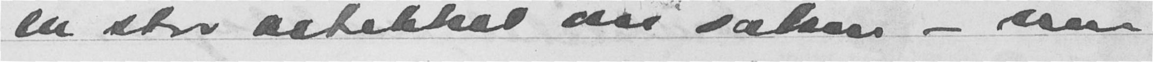en stor artikkel om saken - men
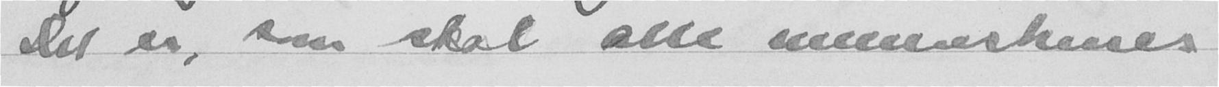Det er, som skal alle menneskenes
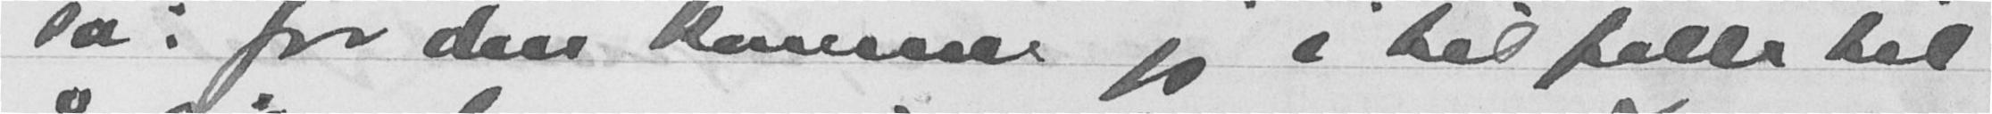sa: for den kommer jeg i tilfelle til
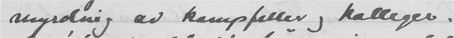myrding av kampfeller og kolleger.
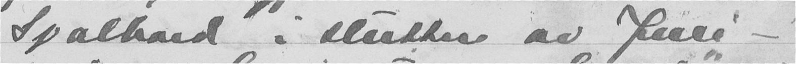Svalbard i slutten av Juli -
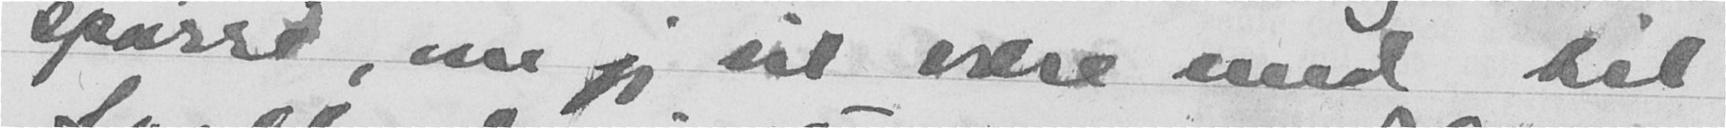spørre, om jeg vil være med til
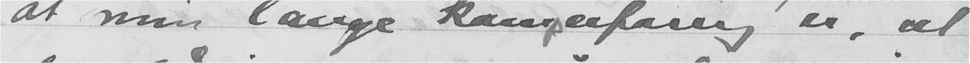at min lange kamperfaring er, at
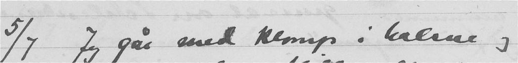5/7 Jeg går med klump i halsen og
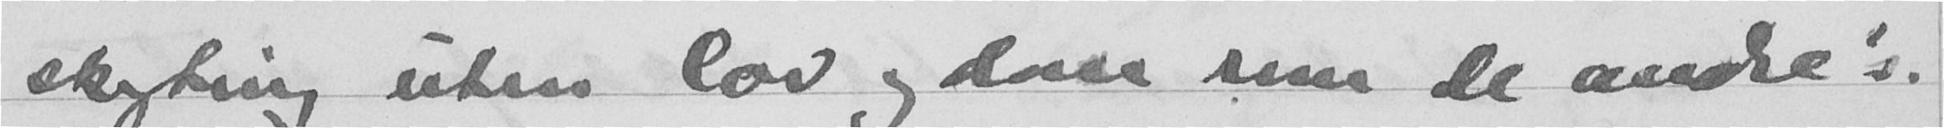skyting uten lov og dosse som de andre:
